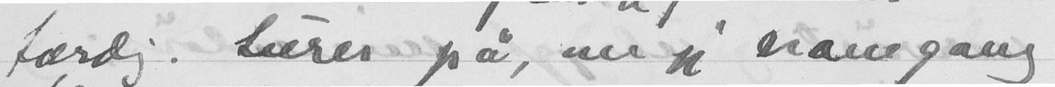færdig. Lurer på, om jeg noengang
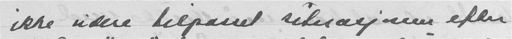ikke videre tilpasset situasjonen efter
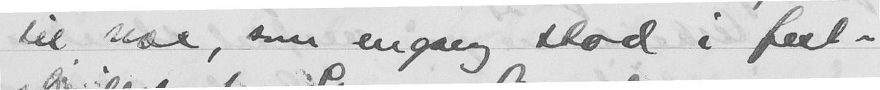til noe, som engang stod i fest-
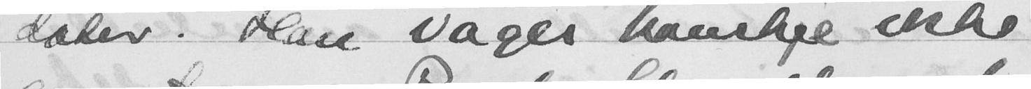doter. Han våger kanskje ikke
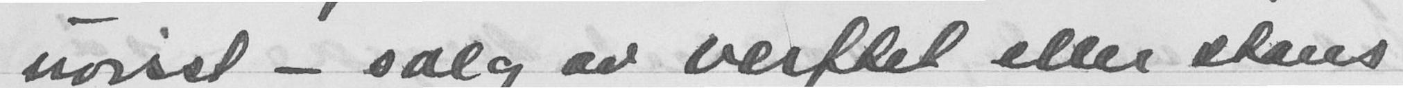uvisst - salg av verftet eller stans
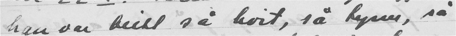han var blitt så hvit, så tynn, så
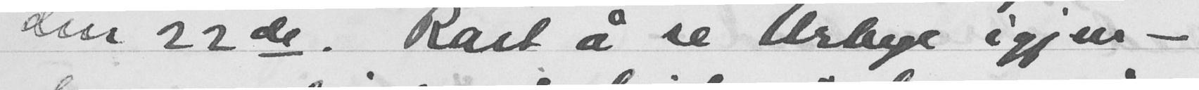den 22de. Rart å se Arbye igjen -
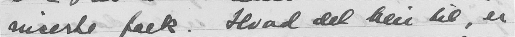niserte folk. Hvad det blir til, er
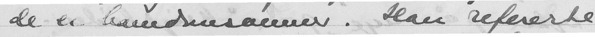de er barndomsvenner. Han refererte
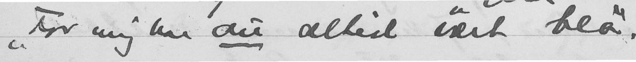"For mig har du altid vært blå".
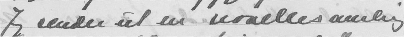Jeg sender ut en novellesamling
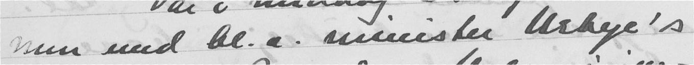men med bl. a. minister Arbye's
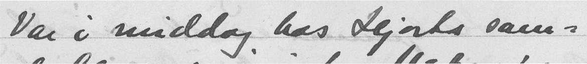Var i middag hos Hjorts sam-
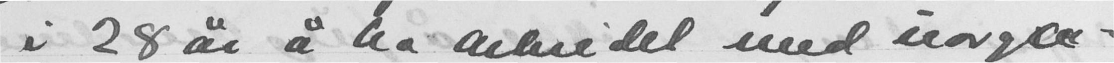i 28 år å ha arbeidet med uorga-
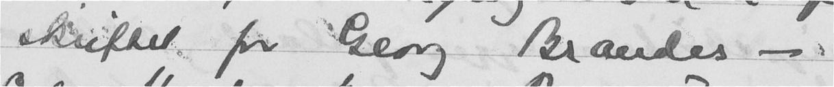skriftet for Georg Brandes -
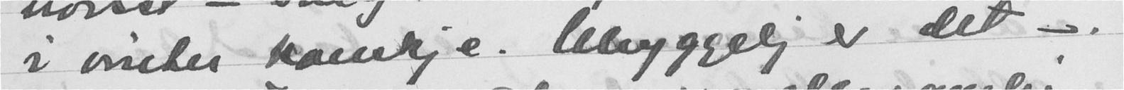i vinter kanskje. Uhyggelig er det -.
